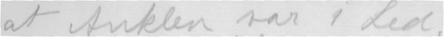at Anklen var i Led,
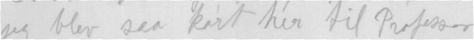Jeg ble saa kørt her til Professor
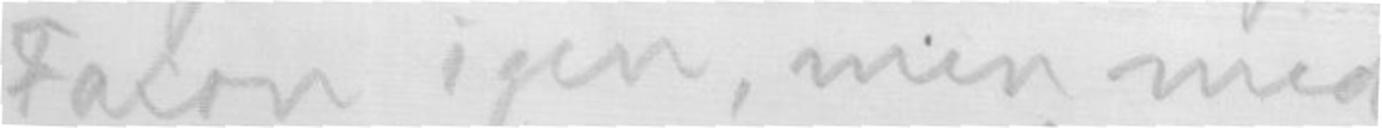Facon igen, men med
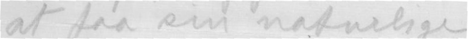at faa sin naturlige
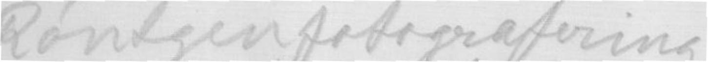Røntgenfotografering.
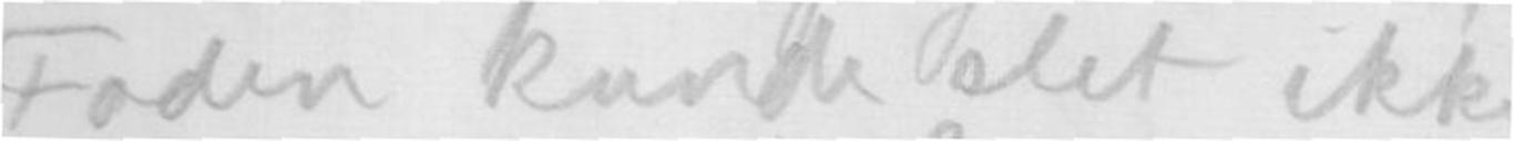Foden kunde slet ikke
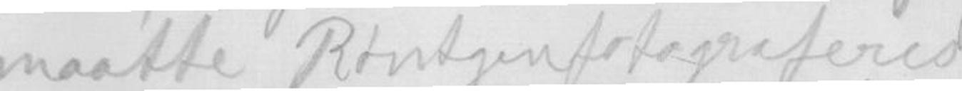maatte Røntgenfotograferes,
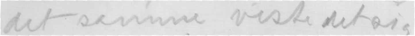det samme viste det sig
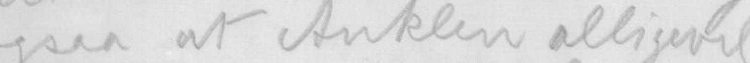ogsaa at Ankelen alligevel
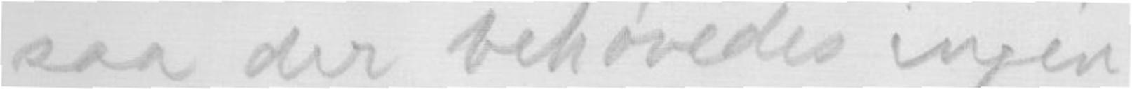saa der behøvedes ingen
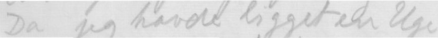Da jeg havde ligget en Uge
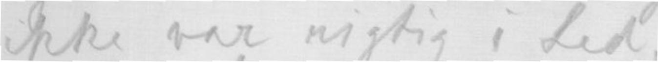ikke var rigtig i led,
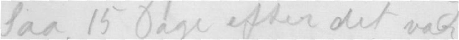Saa, 15 Dage efter det var
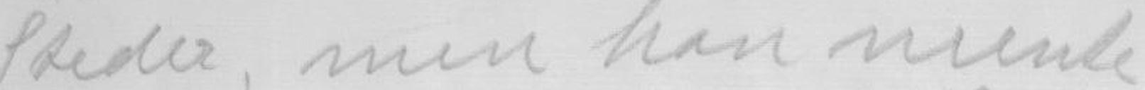Steder, men han mente
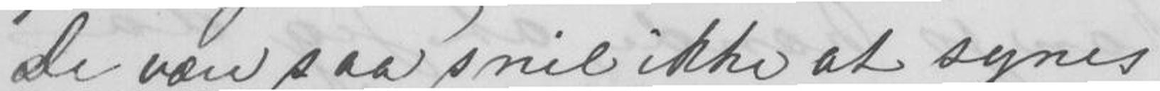De være saa snil ikke at synes
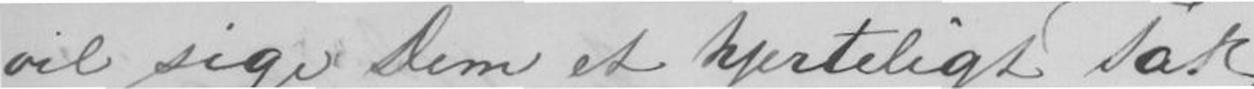vil sige Dem et hjerteligt Tak
